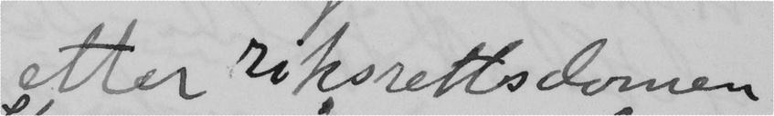etter riksrettsdomen
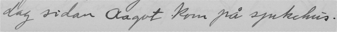dag sidan Aagot kom på sjukehus.
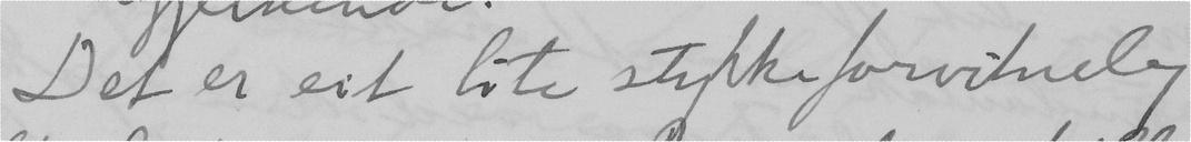Det er eit lite stykke forvitneleg
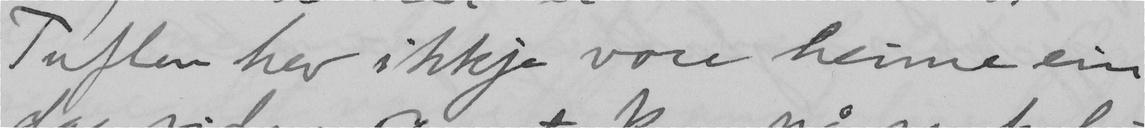Tuften hev ikkje vore heime ein
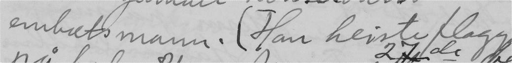embætsmann. (Han heiste flagg
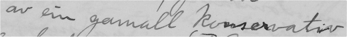av ein gamall konservativ
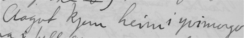Aagot kjem heim i yvimorgo
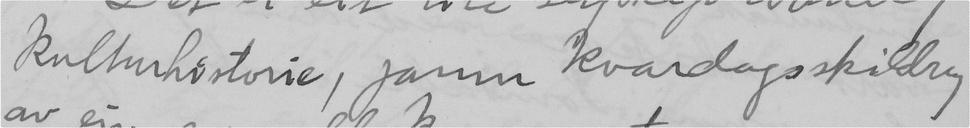kulturhistorie, jamn kvardagsskildring
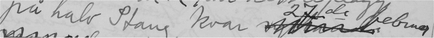på halv Stang kvar 27de februar
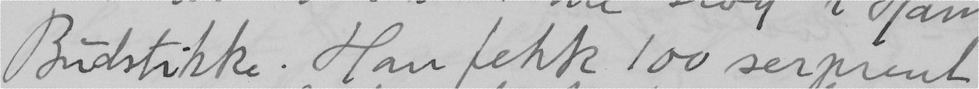Budstikke. Han fekk 100 serpient
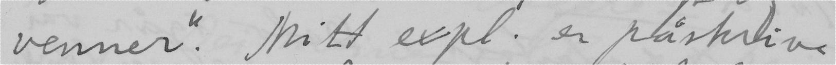venner". Mitt expl. er påskrive
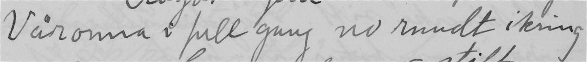Våronna i full gang no rundt ikring
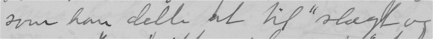som han delte ut til "slægt og
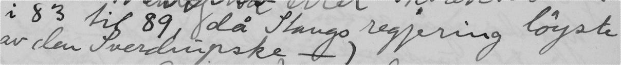i 83 til 89, då Stangs regjering löyste
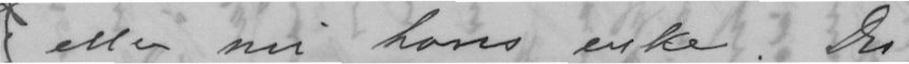eller nu hans enke. Du
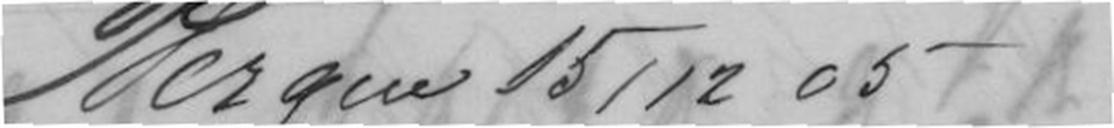Bergen 15/12 05
